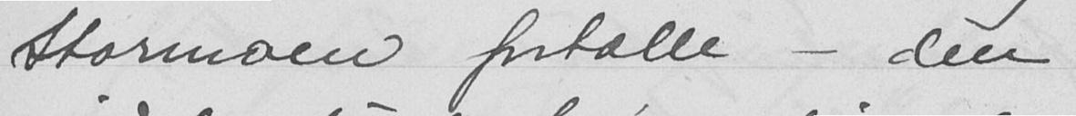Stormoen fortælle - den
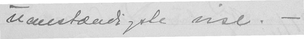uanstændigste vise. -
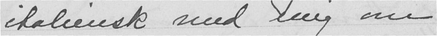italiensk med mig om
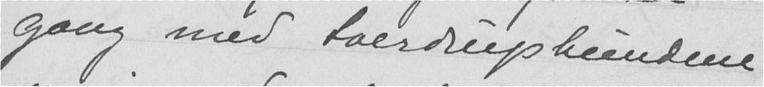gang med Sverdrupshundene
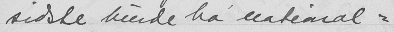sidste burde ha national-
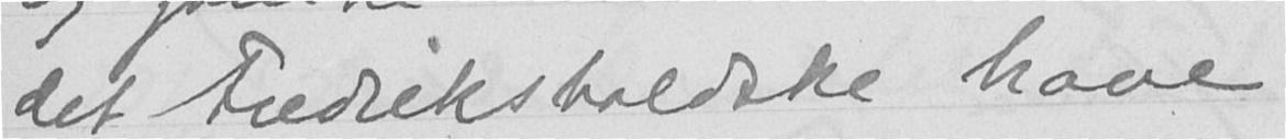det Fredrikshaldske have
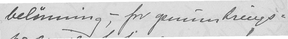belønning, - for opmuntrings-
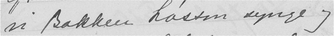vi Bokken Lasson synge og
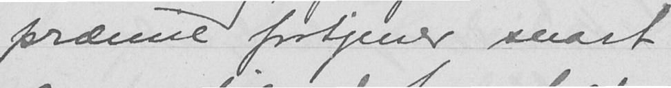præmie fortjener snart
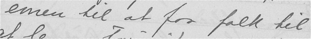evnen til at faa folk til
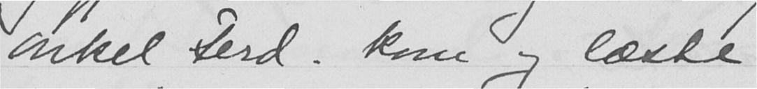Onkel Ferd. kom og læste
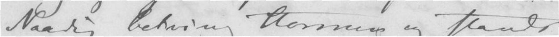Naadig betving Stormens og stands
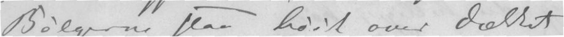Bølgerne slaa høit over dækket,
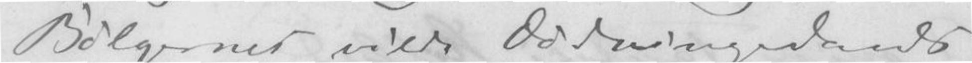Bølgernes vilde dødningedans!
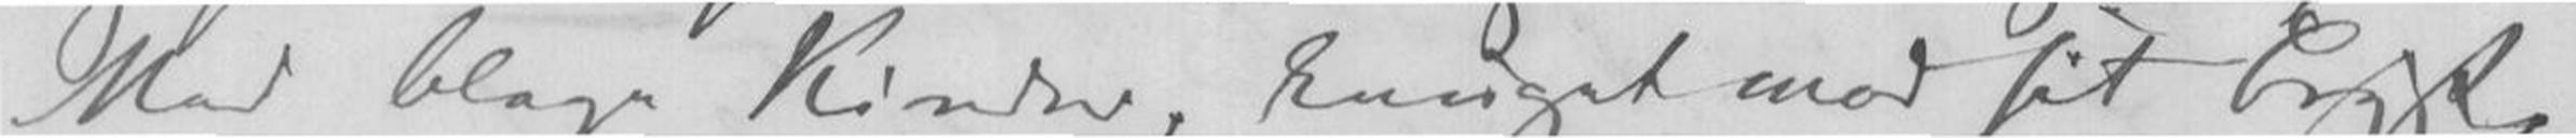Med blæge Kinder, knuget mod sit Bryst,.
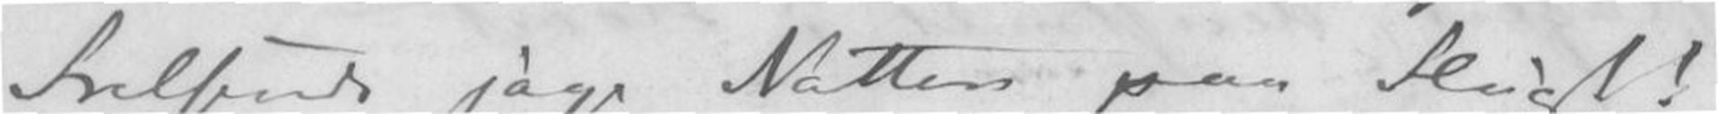Frelsende jage Natten paa Flugt!
